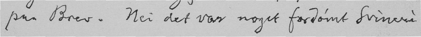paa Brev. Nei det var noget fordømt Svineri
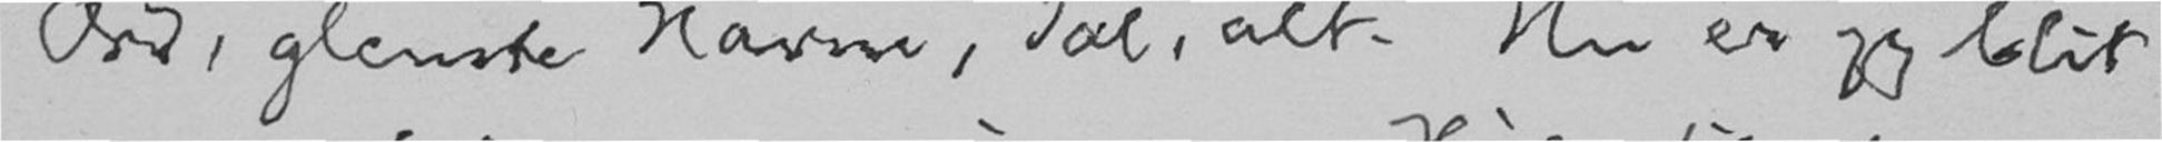Ord, glemte Navne, Tal, alt. Nu er jeg blit
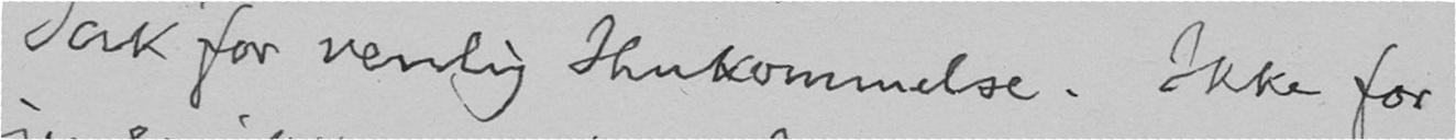Tak for venlig Hukommelse. Ikke for
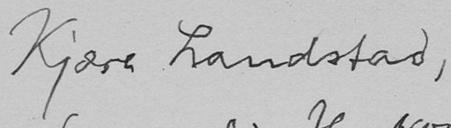Kjære Landstad,
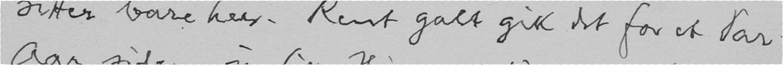sitter bare her. Rent galt gik det for et Par
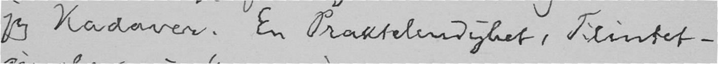jeg Kadaver. En Praktelendighet, Tilintet-
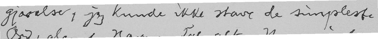gjørelse, jeg kunde ikke stave de simpleste
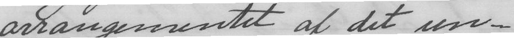arrangementet af det un-
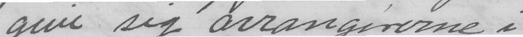give sig arrangørerne i
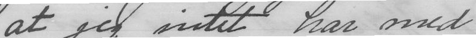at jeg intet har med
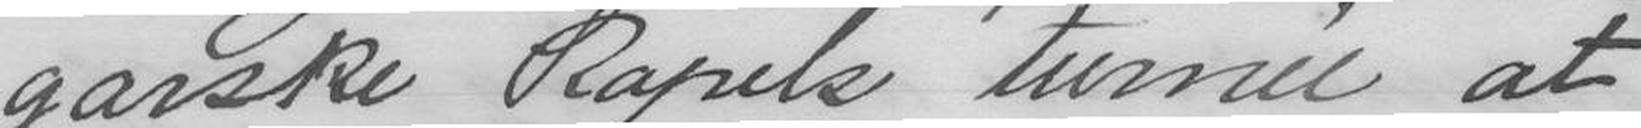garske Rapels turnée at
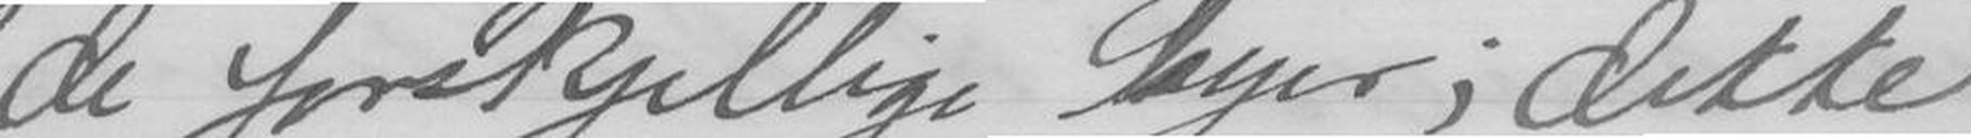de forskjillige byer; dette
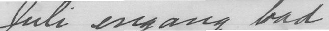Juli engang bad
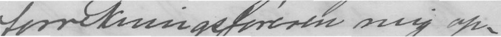foretningsføreren mig op-
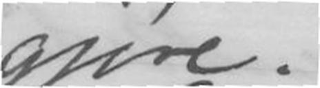gjøre.
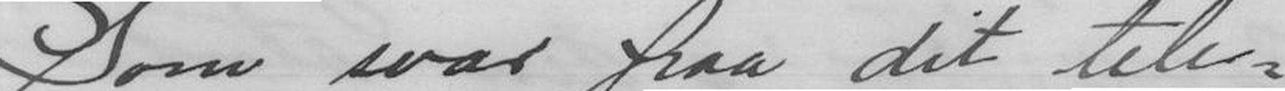Som svar paa dit tele-
